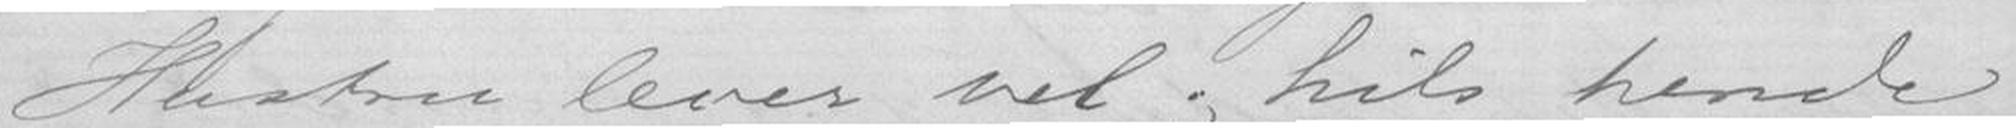Hustru lever vel; hils hende
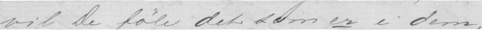vil De føle det som er i dem,
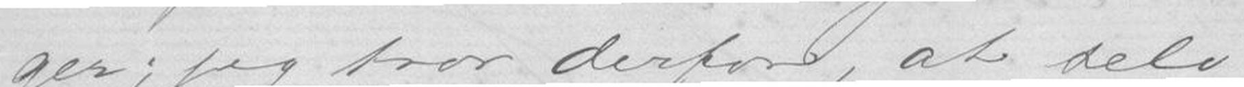ger; jeg tror derfor, at selv
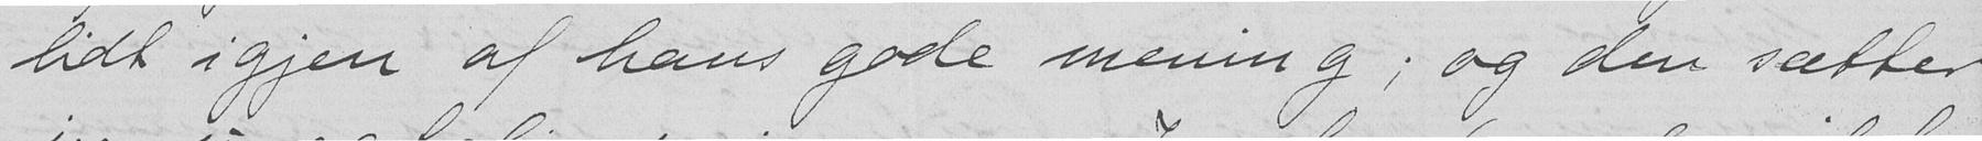lidt igjen af hans gode mening; og den sætter
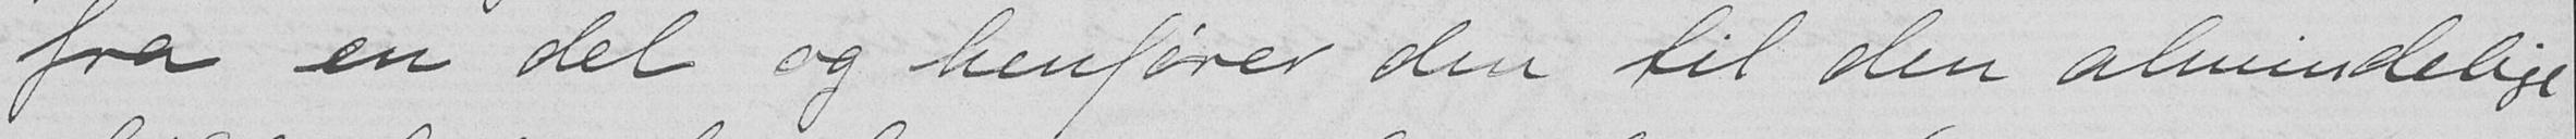fra en del og henfører den til den almindelige
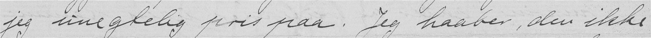jeg unegtelig pris paa. Jeg haaber, den ikke
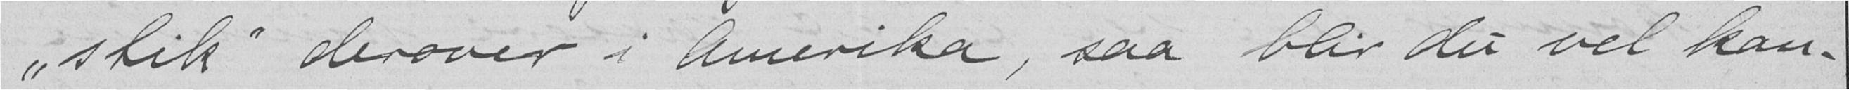"stik" derover i Amerika, saa blir du vel kan-
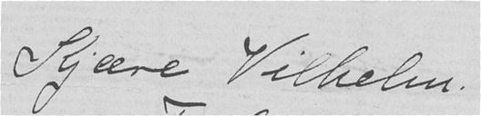Kjære Vilhelm!
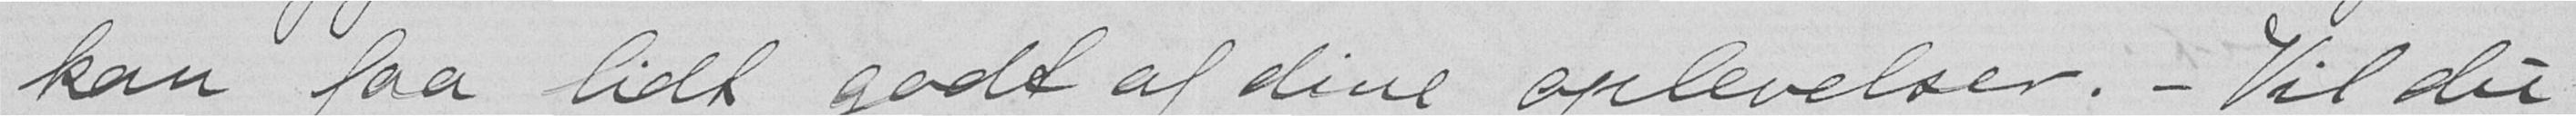kan faa lidt godt af dine oplevelser. – Vil du
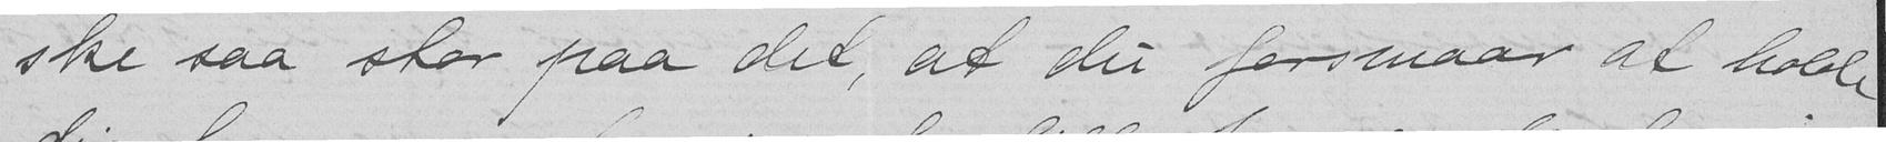ske saa stor paa det, at du forsmaar at holde
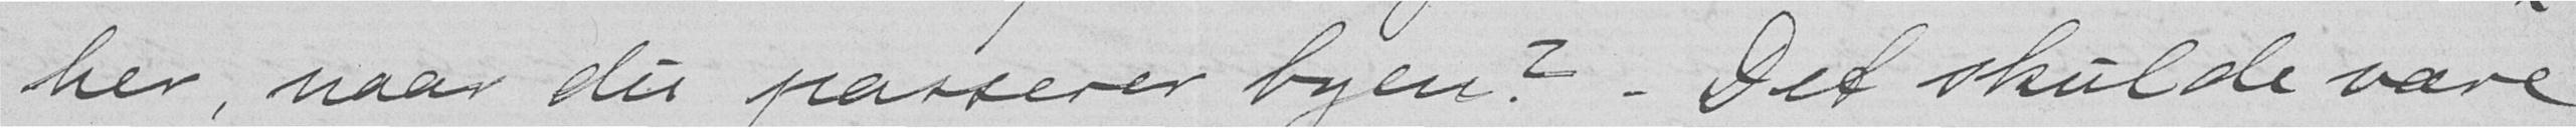her, naar du passerer byen? – Det skulde være
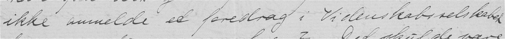ikke anmelde et foredrag i Videnskabsselskabet
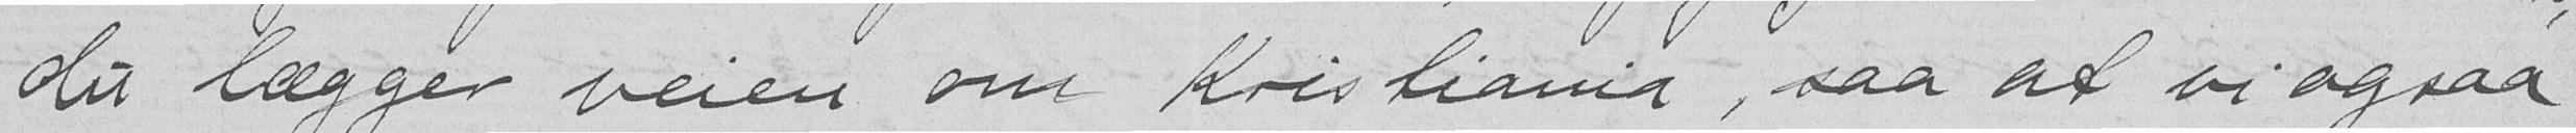du lægger veien om Kristiania, saa at vi ogsaa
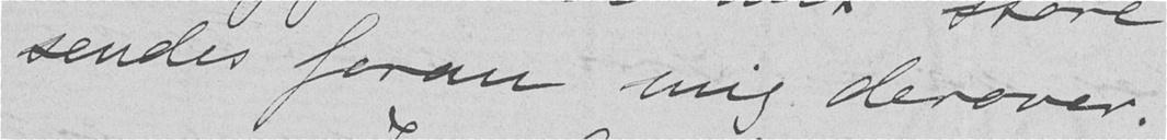sendes foran mig derover.
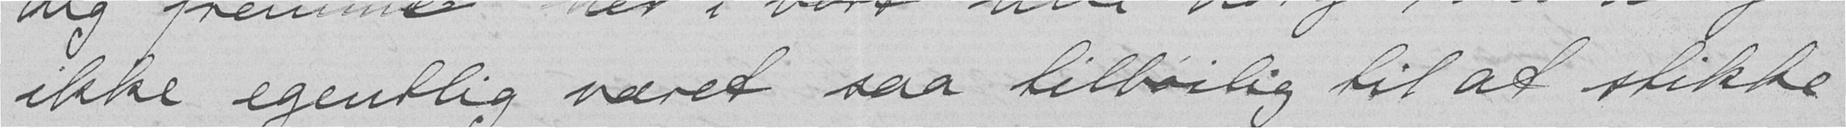ikke egentlig været saa tilbøielig til at stikke
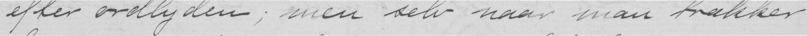efter ordlyden; men selv naar man trækker
